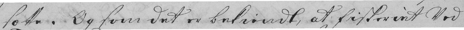sætte. Og som det er bekiendt, at Fiskeriet Ved
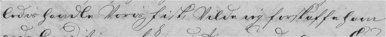ledis handle Vores Fisk, Vilde ieg forskaffe ham
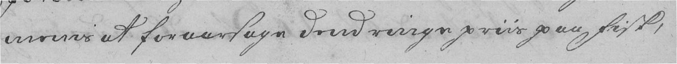menis at foraarsage dend ringe priis paa Fisk,
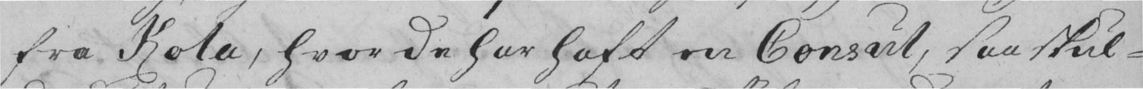fra Kola, hvor de har haft en Consul, saa skul-
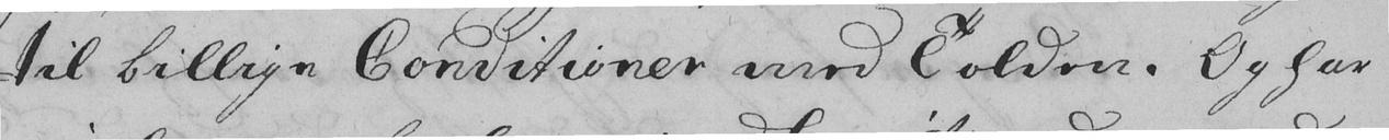til billige Conditioner med Tolden. Og har
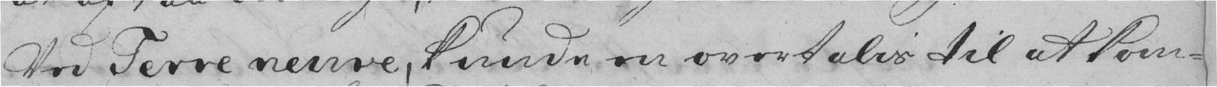Ved Terre neuve, kunde en overtalis til at kom-
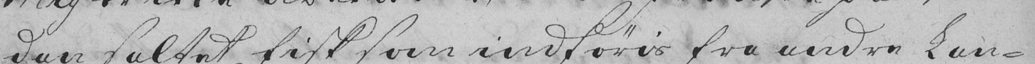dan saltet Fisk som indføris fra andre Lan-
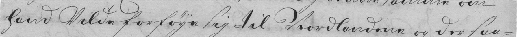hand Vilde forføye sig til Nordlandene og der saa-
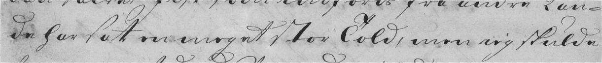de har sat en meget stor Told, men ieg skulde
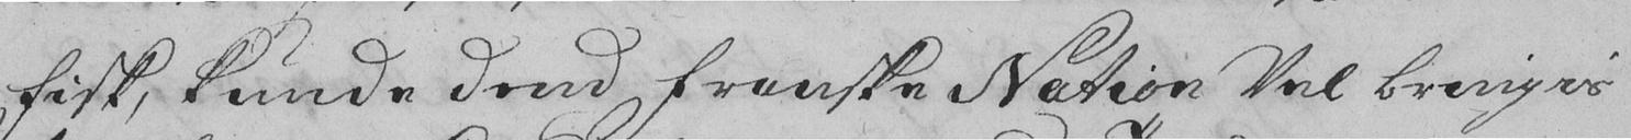Fisk, kunde dend Franske Nation vel bringis
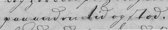paa anden tid og stæd.
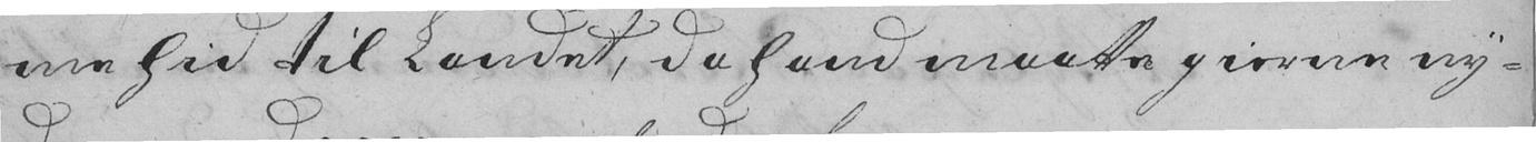me hid til Landet, da hand maatte gierne ny-
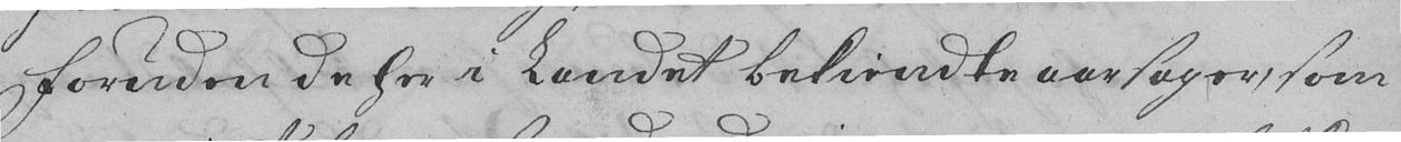Foruden de her i Landet bekiendte aarsager, som
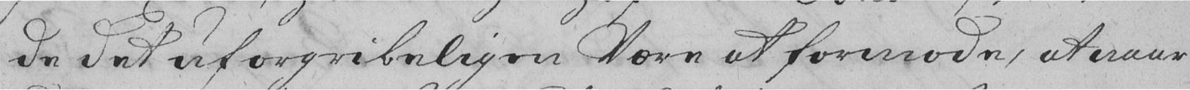de det uforgribeligen være at formode, at naar
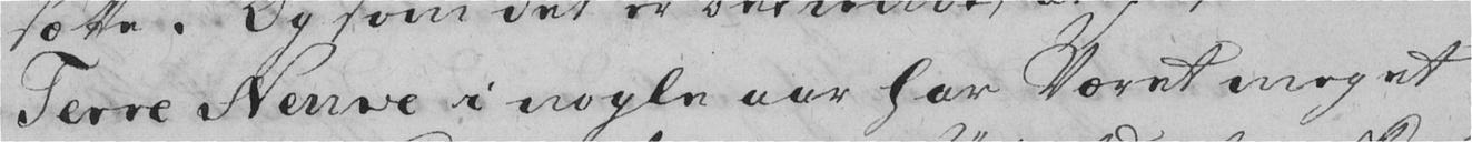Terre Neuve i nogle aar har Været meget
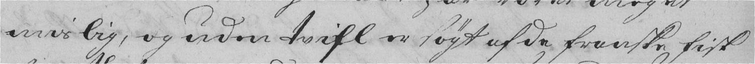mislig, og uden tvifl er søgt af de Franske Fisk
